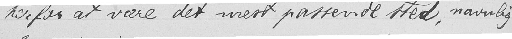herfor at være det mest passende sted, navnlig
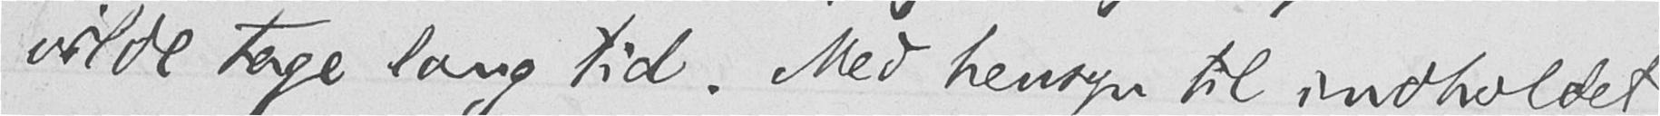vilde tage lang tid. Med hensyn til indholdet,
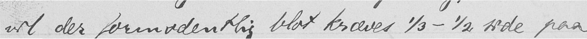vil der formodentlig blot kræves 1/3 - 1/2 side paa
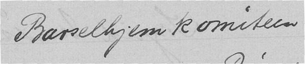Barselhjemkomiteen
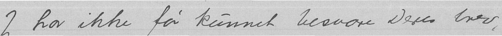Jeg har ikke før kunnet besvare Deres brev
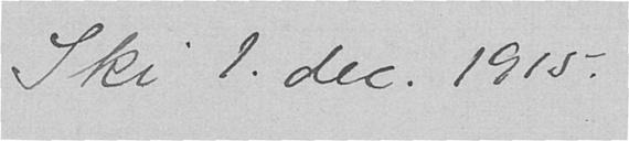Ski 1. dec. 1915.
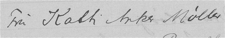Fru Katti Anker Møller
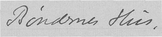Bøndernes Hus,
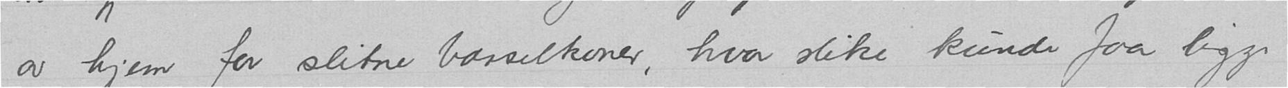av hjem for slitne barselkoner, hvor slike kunde faa ligge
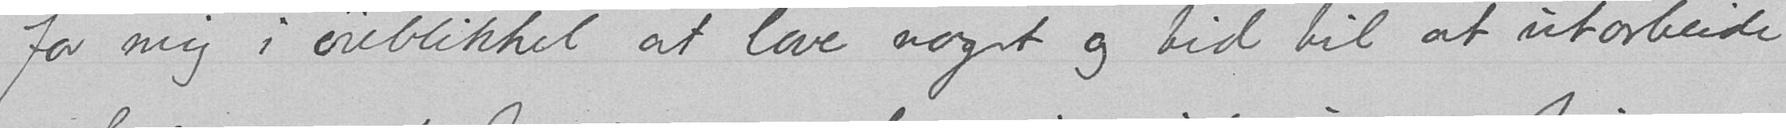for mig i øieblikket at lave noget og tid til at utarbeide
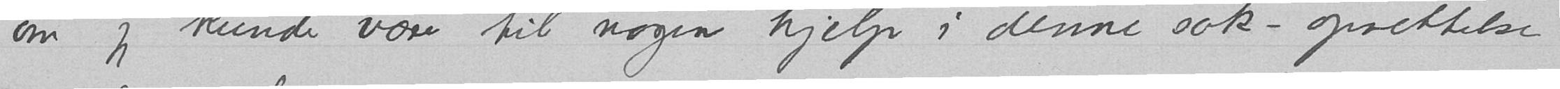om jeg kunde være til nogen hjelp i denne sak - oprettelse
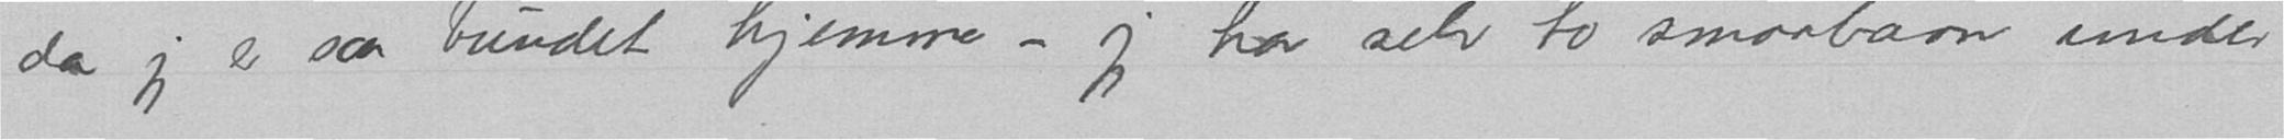da jeg er saa bundet hjemme – jeg har selv to smaabarn under
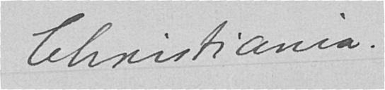Christiania.
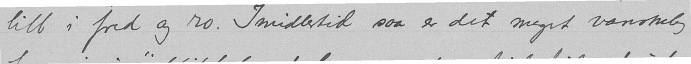litt i fred og ro. Imidlertid saa er det meget vanskelig
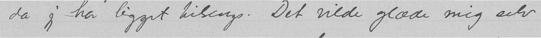da jeg har ligget tilsengs. Det vilde glæde mig selv
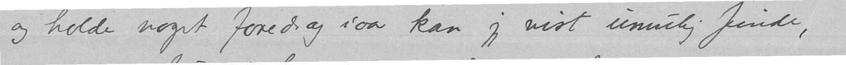og holde noget foredrag iaar kan jeg vist umulig finde,
